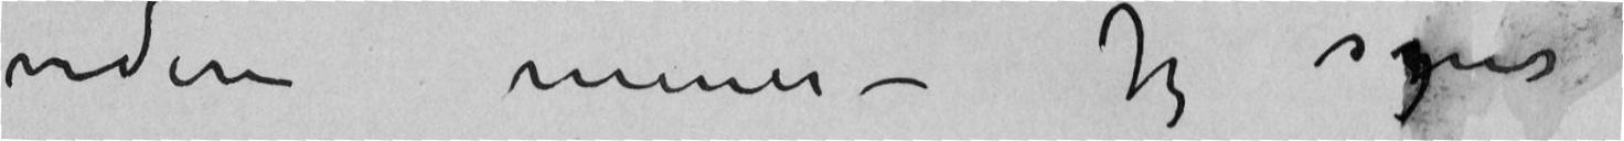videre mener – Jeg syns
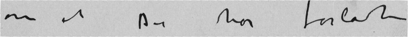om at du har forladt
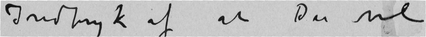Indtryk af at du vil
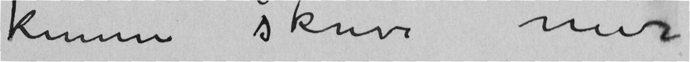kunne skrive mer
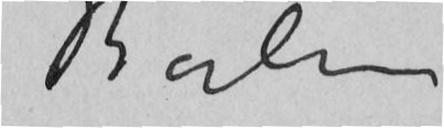Berlin
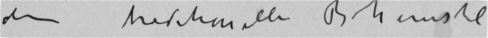den tradisionelle Bohemstil
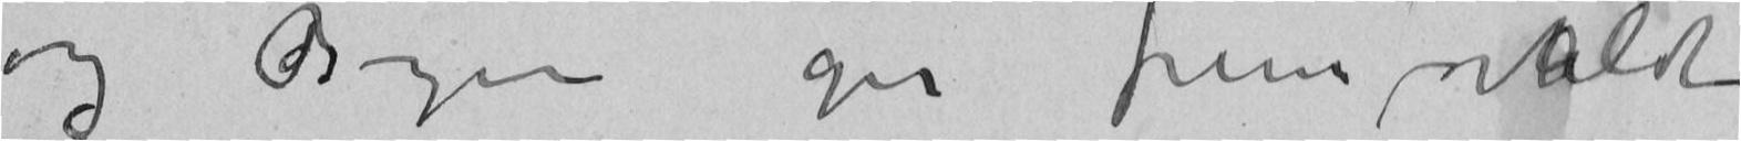og Bogen gir fremforalt
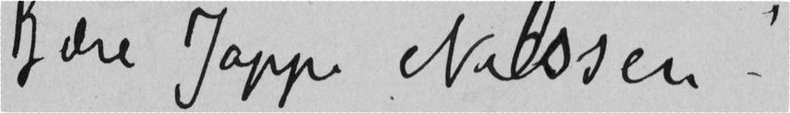Kjære Jappe Nilssen!
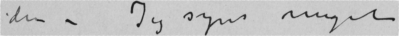den – Jeg syns meget
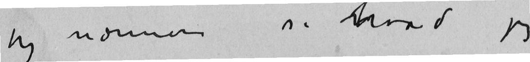jeg nærmere si hvad jeg
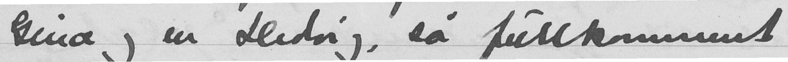sma og en Hedvig, sa fullkomment
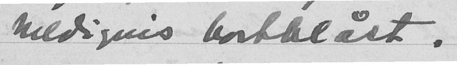heldigens bortblåst.
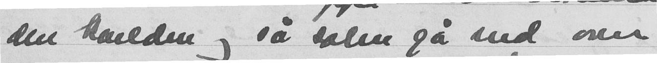den kvelden og så salen gå ind over
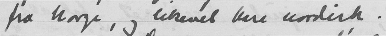fra Norge likevet bare norderk.
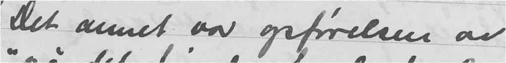Det annet var opførelsen av
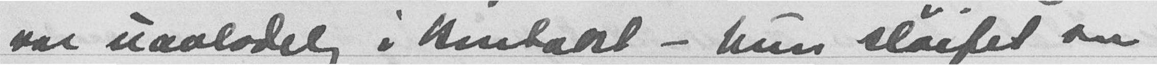var uavladelig i kontakt - hun sløifet som
